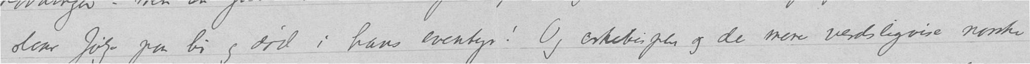slaar følge paa liv og død i hans eventyr! Og erkebispen og de mere verdsligvise norske
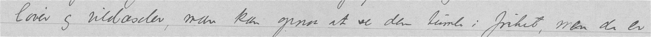løver og vildæseler, man kan opnaa at se den tumle i frihet, men de er
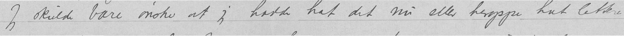Jeg skulde bare ønske at jeg hadde hat det nu eller heroppe hat lettere
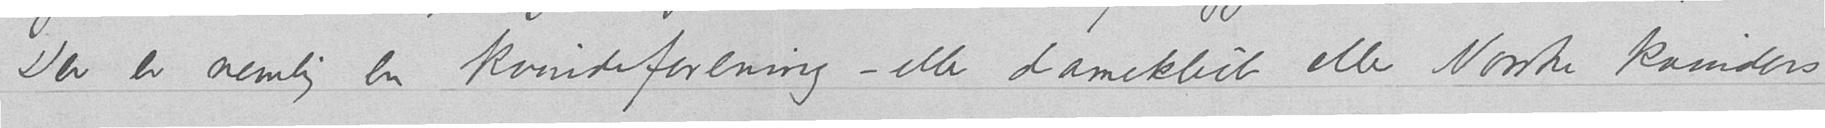Der er nemlig en kvindeforening – eller dameklub eller Norske Kvinders
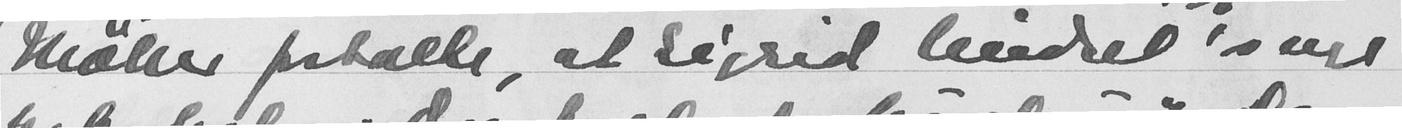Møller fortalte, at Sigrid Undset's nye
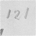121
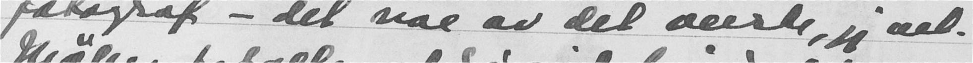fotograf - det noe av det verste, jeg vet.
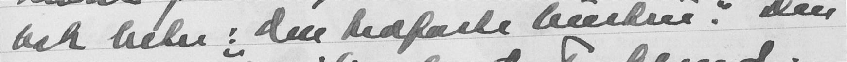bok heter: "den trofaste hustru." Den
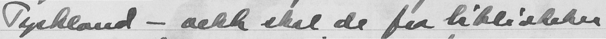Tyskland - vekk skal de fra biblioteker
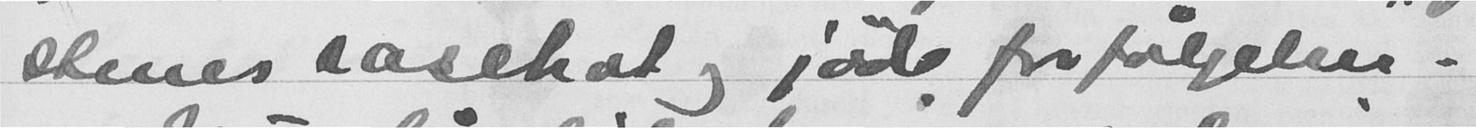stenes rasehat og jødeforfølgelser.
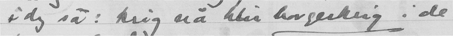idag sa: krig nå blir borgerkrig i de
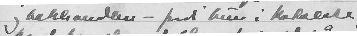og bokhandlere - fred kun i katolske
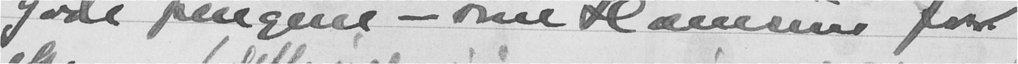gode pengene - som Hamsun for
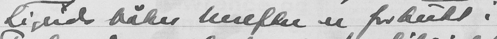Sigrids bøker herefter er forbutt i
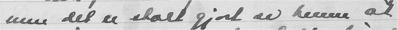men det er stolt gjort av henne at
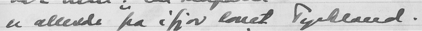er allerde fra ifjor lovet Tyskland.
